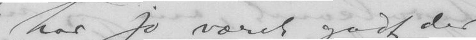har jo været godt der
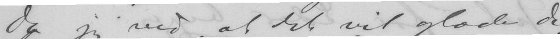Da jeg ved, at det vil glæde dig,
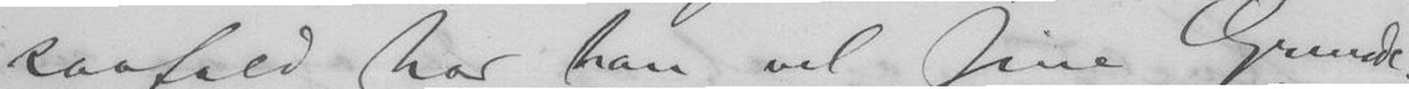saafald har han vel sine Grunde.
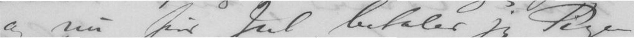og nu før Jul betaler jeg Pige
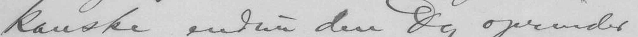kanske endnu den Dag oprinder,
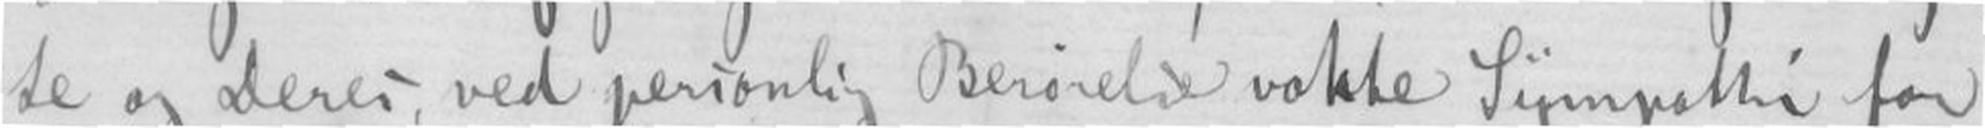te og Deres, ved personlig Berøvelse vakte Sympathi for
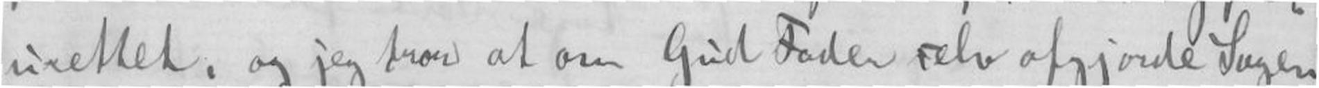urettet, og jeg tror at om Gud Fader selv afgjorde Sagen
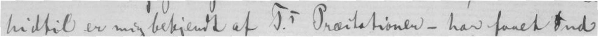hidtil er mig bekjendt af T.s Præstationer - har faaet Ind
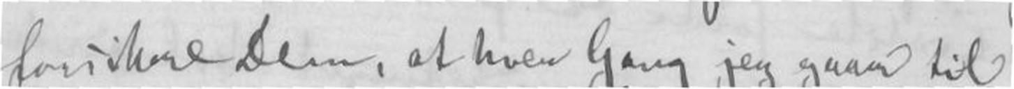forsikre Dem, at hver Gang jeg gaar til
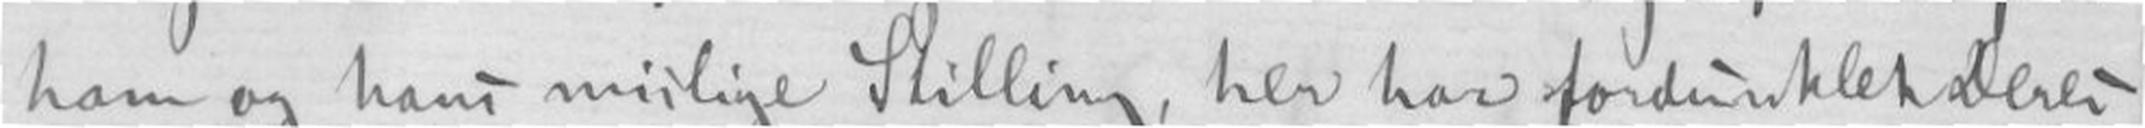ham og hans mislige Stilling, her har fordunklet Deres
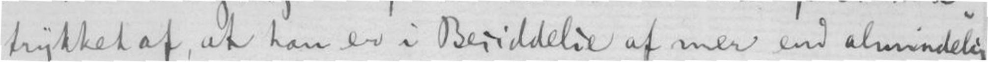trykket af, at han er i Besiddelse af mer end almindelig
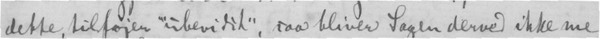dette, tilføjer "ubevidst", saa bliver Sagen derved ikke me
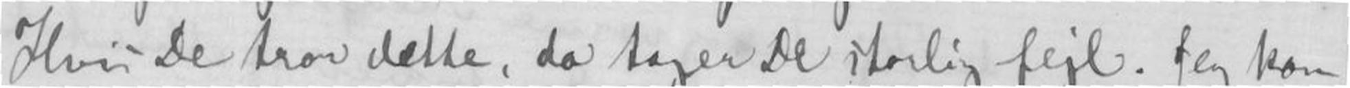Hvis De tror dette, da tager De storlig fejl. Jeg kan
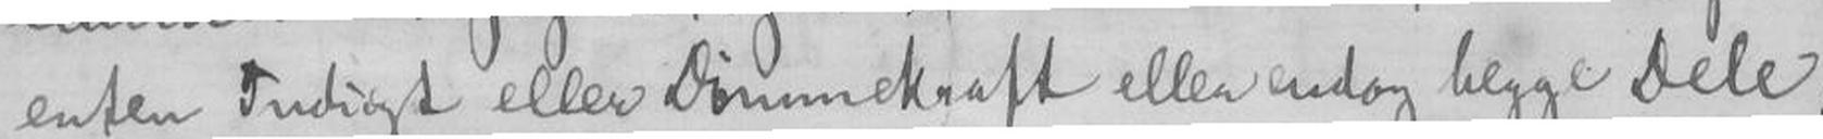enten Insigt eller Drømmekraft eller endog begge Dele,
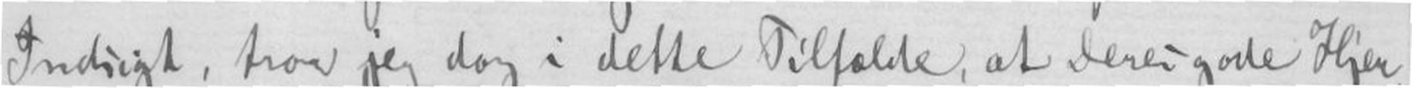Indsigt, tror jeg dog i dette Tilfælde, at Deres gode Hjer
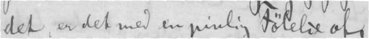det, er det med en pinlig Følelse af
